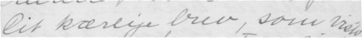Dit kærlige brev, som viste
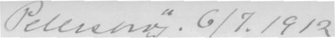Petershøj. 6/7. 1912
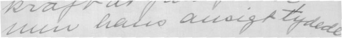men hans ansigt tydede
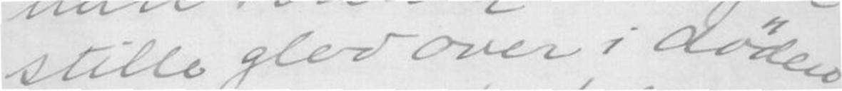stille gled over i døden
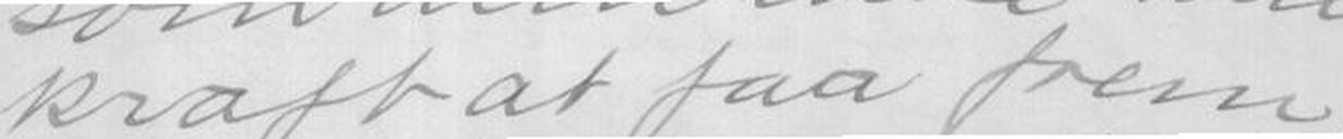kraft at faa frem
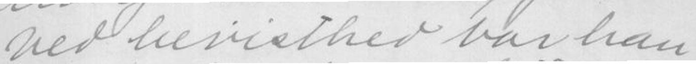ved bevisthed var han
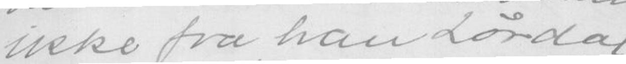ikke fra han Lørdag
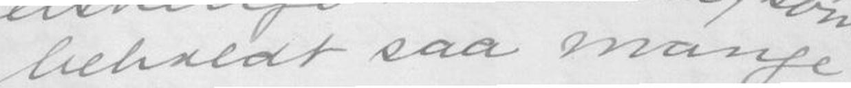beholdt saa mange
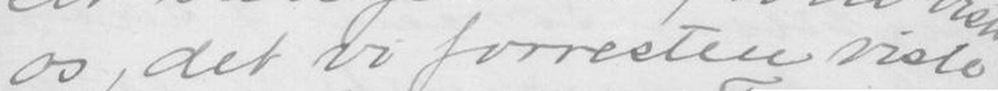os, det vi forresten viste
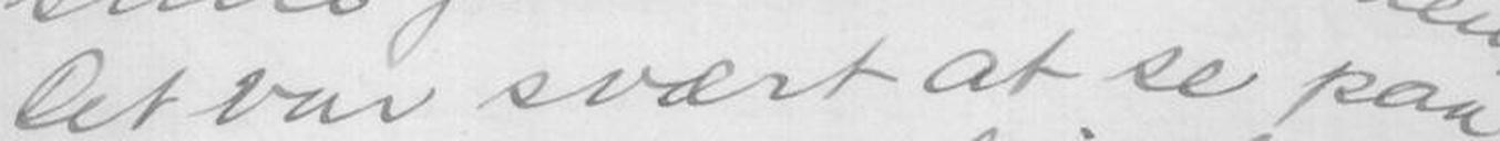Det var svært at se paa
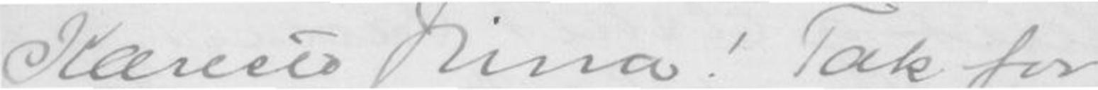Kæreste Nina! Tak for
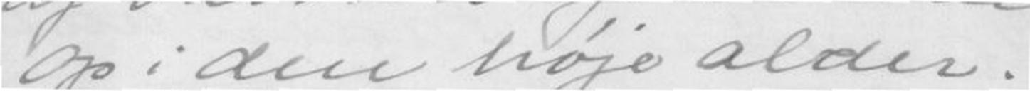op i den høje alder.
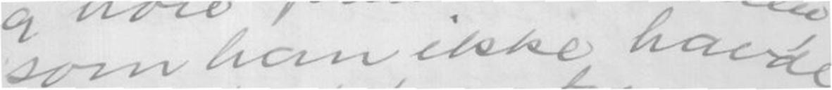som han ikke havde
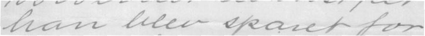han blev sparet for
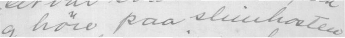og høre paa slimhosten
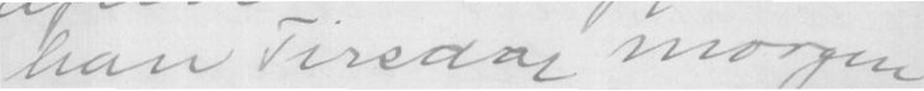han Tirsdag morgen
